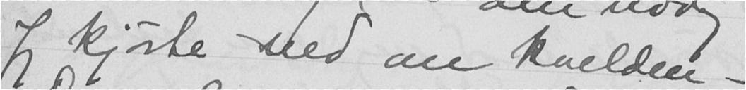Jeg kjørte ned om kvelden -
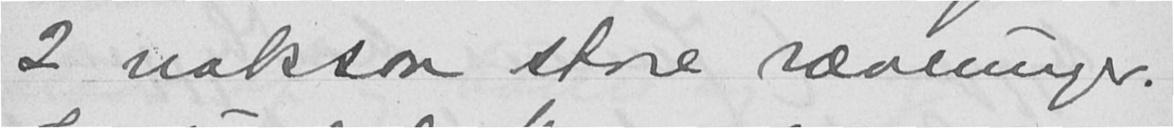2 noksaa store ræveunger.
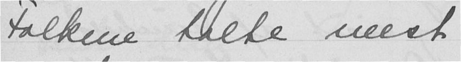Folkene talte mest
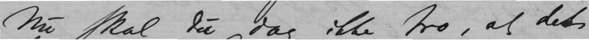Nu skal du dog ikke tro, at det
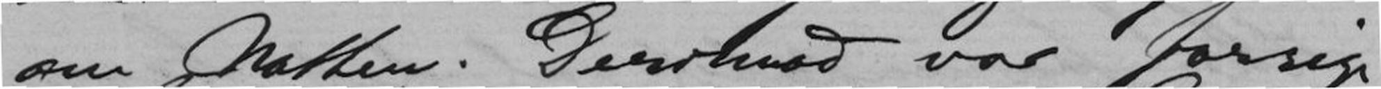om Natten. Derimod var forrige
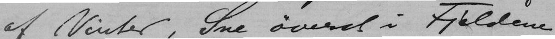af Vinter, Sne øverst i Fjeldene,
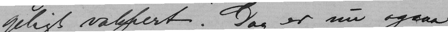geligt vakkert. Dog er nu ogsaa
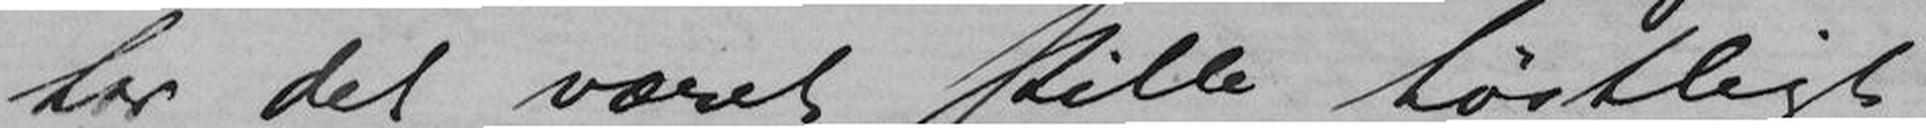har det været stille høstligt
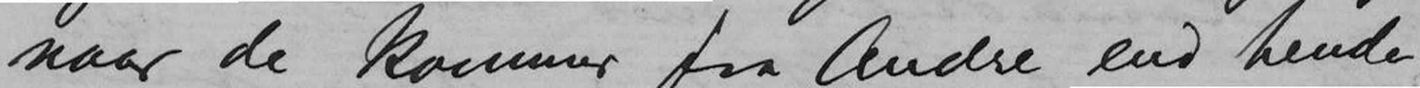naar de kommer fra Andre end hende.
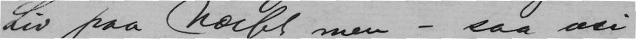Liv paa Næsset, men - saa usi-
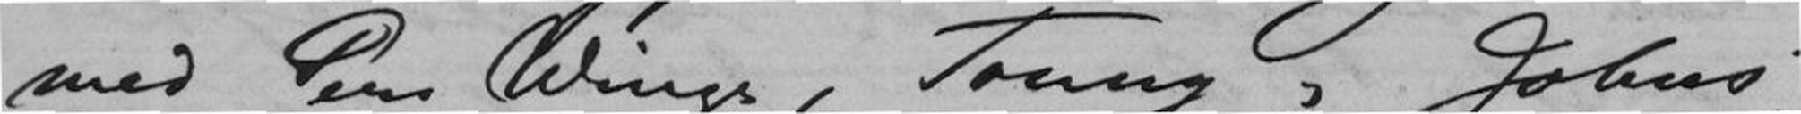med Per Winge, Tonny og Johns, -
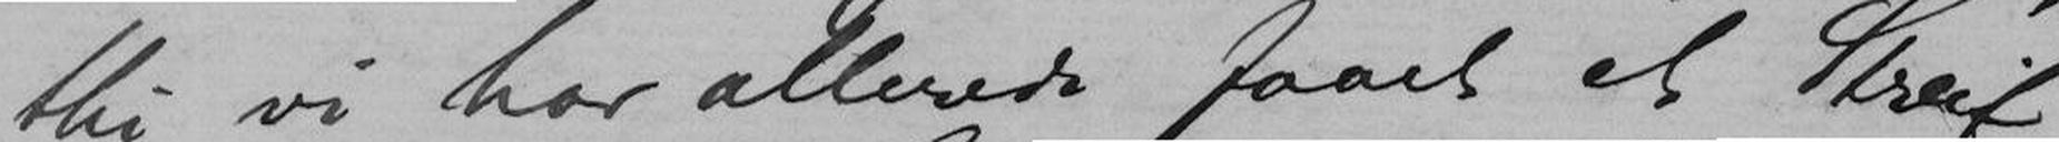thi vi har allerede faaet et Streif
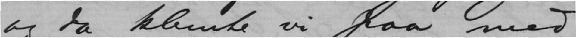og da klemte vi paa med
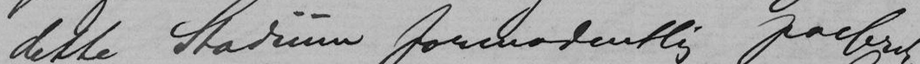dette Stadium formodentlig passeret,
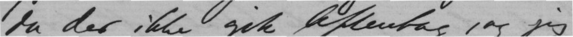da der ikke gik Aftentog, og jeg
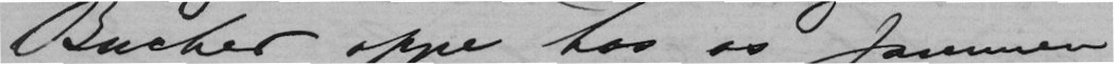Bucher oppe hos os sammen
